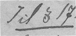Til § 17.
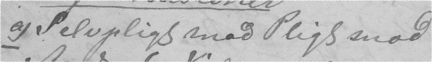a) Selvpligt mod Pligt mod
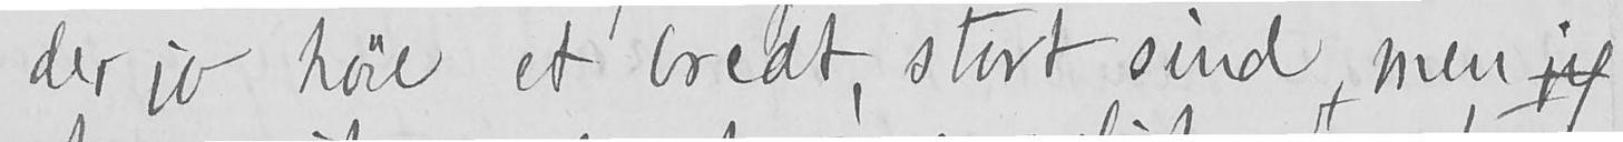der jo høre et bredt, stort sind, men jeg
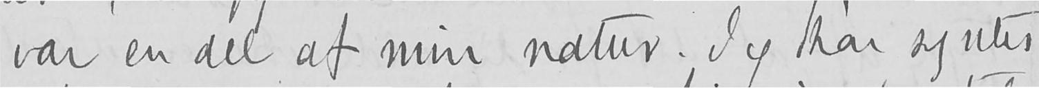var en del af min natur. Jeg har syntes
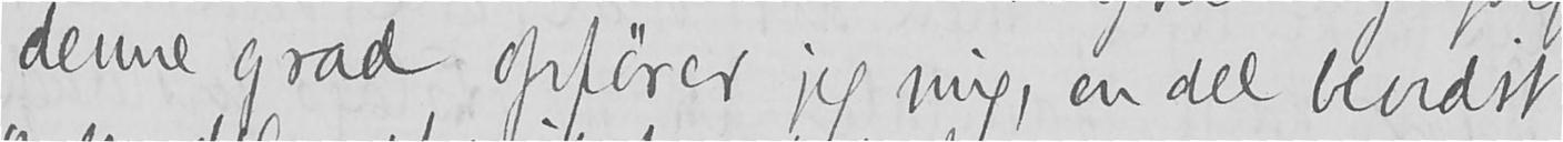denne grad opfører jeg mig, en del bevidst
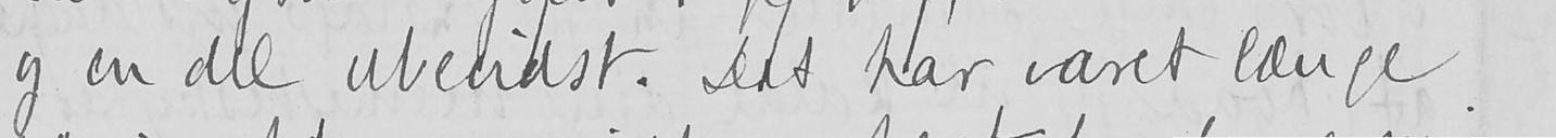og en del ubevidst. Det har varet længe
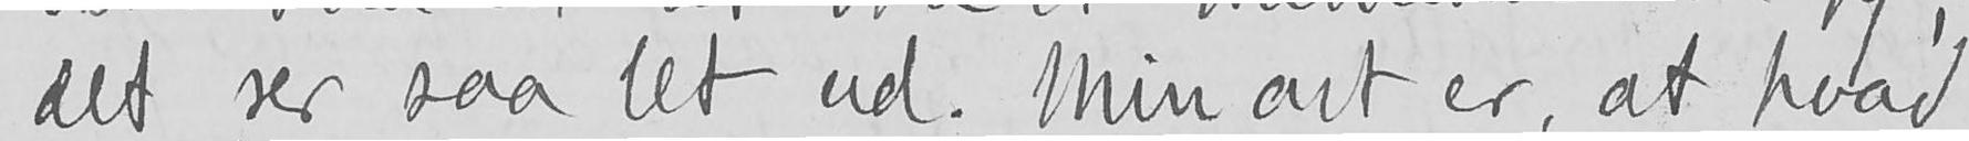det ser saa let ud. Min art er, at hvad
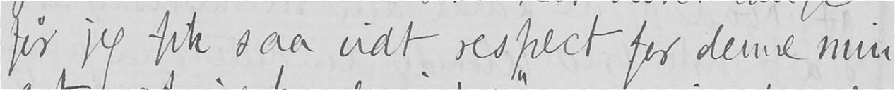før jeg fik saa vidt respect for denne min
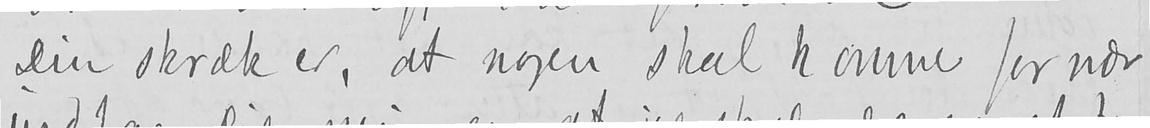Din skræk er, at nogen skal komme for nær
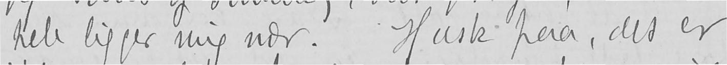hele ligger mig nær. Husk paa, det er
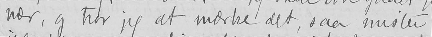nær, og tror jeg at mærke det, saa mister
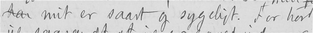har mit er saart og sygeligt. For tror
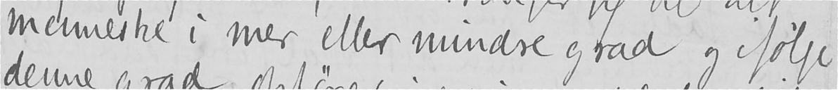menneske i mer eller mindre grad og ifølge
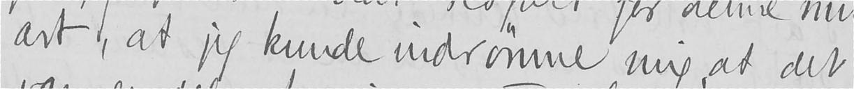art, at jeg kunde indrømme mig, at det
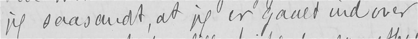jeg saasandt, at jeg er gaaet indover
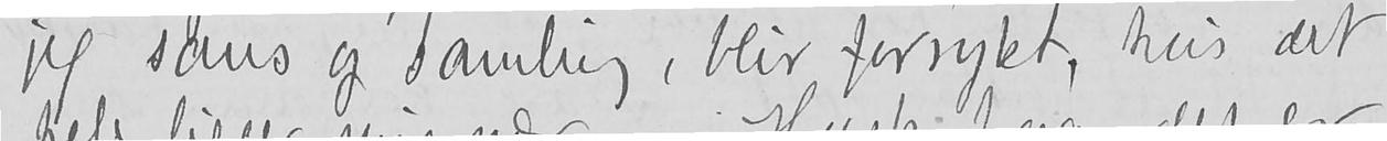jeg sans og samlig, blir forrykt, hvis det
